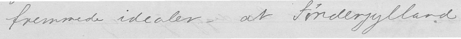fremmede idealer - at Sønderjylland
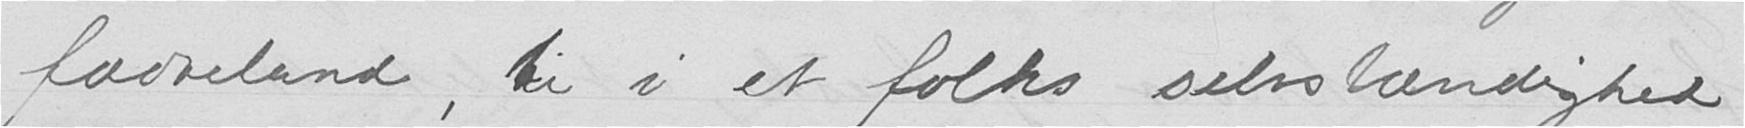fædreland, ti i et folks selvstændighed
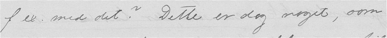f.ex. med det? Dette er dog noget, som
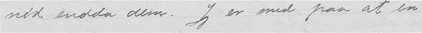nød endda dem. Jeg er med paa at en
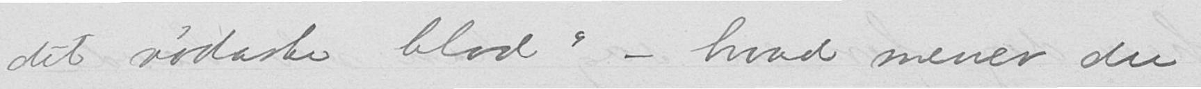det rødaste blod" - hvad mener du
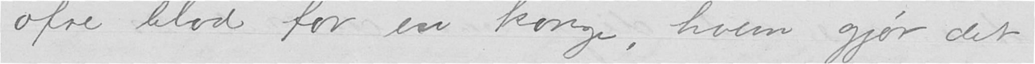ofre blod for en konge, hvem gjør det
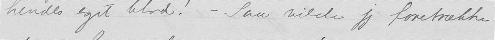hendes eget blod! - Saa vilde jeg foretrække
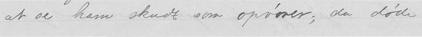at se ham skudt som oprører, da døde
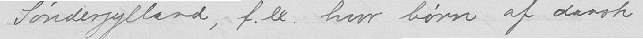Sønderjylland, f.ex. hvor børn af dansk
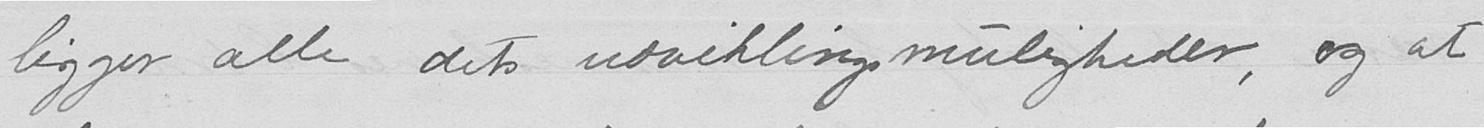ligger alle dets udviklingsmuligheder, og at
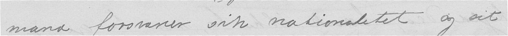mand forsvarer sin nationalitet og sit
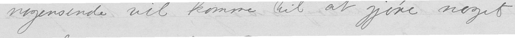nogensinde vil komme til at gjøre noget
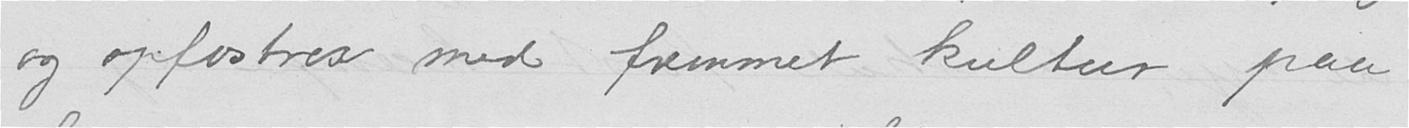og opfostres med fremmet kultur paa
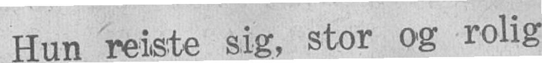Hun reiste sig, stor og rolig
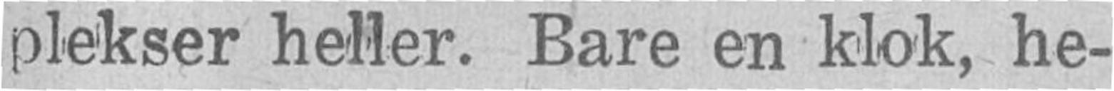plekser heller. Bare en klok, he-
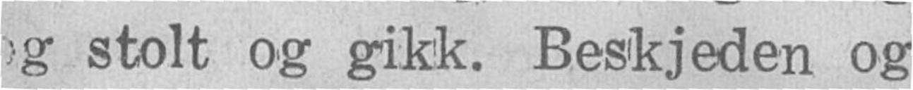og stolt og gikk. Beskjeden og
